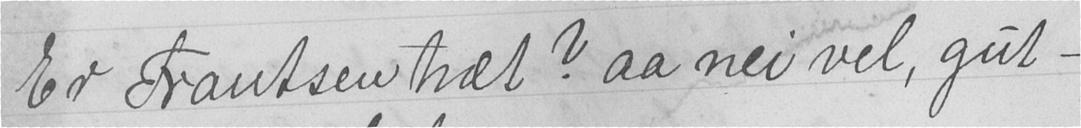Er Frantsen træt? aa nei vel, gut -
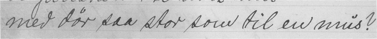med dør saa stor som til en mus?
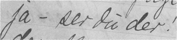ja - ser du der!
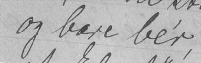og bare be'r,
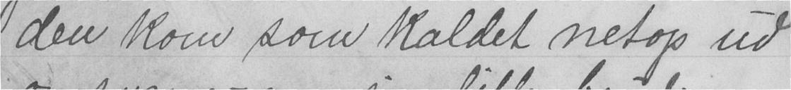den kom som kaldet netop ud
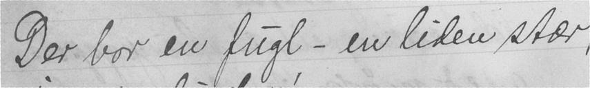Der bor en fugl - en liden stær,
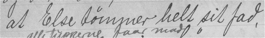at Else tømmer helt sit fad,
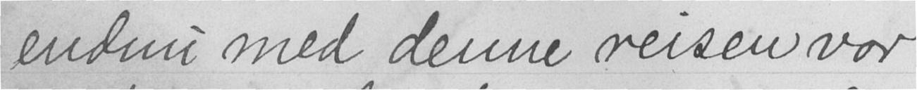endnu med denne reisen vor
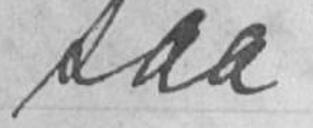saa
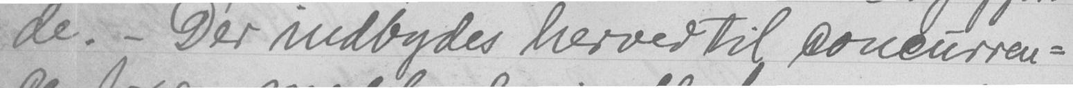de. - Der indbydes herved til concurran-
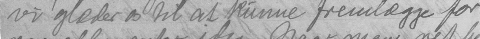vi glæder os til at kunne fremlægge for
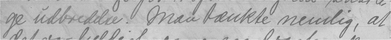ge udbredelse. Man tænkte nemlig, at
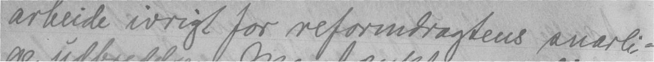arbeide ivrigt for reformdragtens snarli-
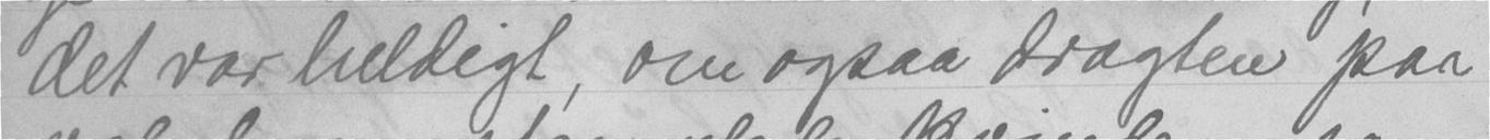det var heldigt, om ogsaa dragten paa
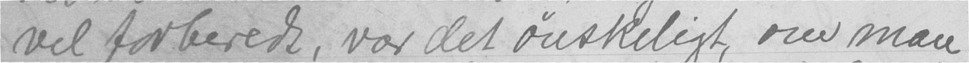vel forberedt, var det ønskeligt, om man
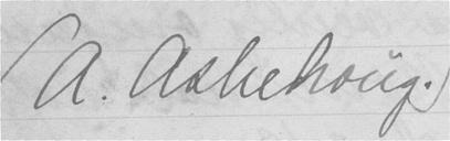(A. Ashehoug.)
